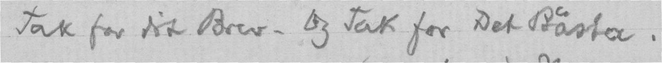Tak for dit Brev. Og Tak for Det Bästa.
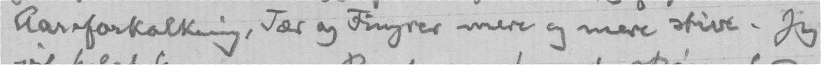Aareforkalkning, Tær og Fingrer mere og mere stive. Jeg
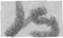jeg
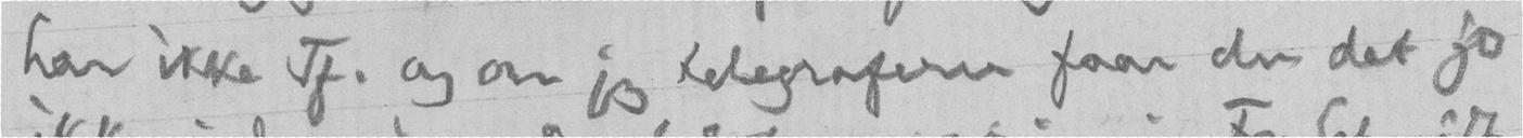har ikke Tf. og om jeg telegraferer faar du det jo
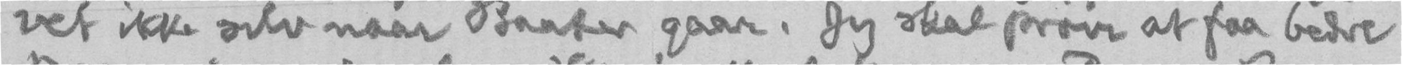vet ikke selv naar Baater gaar. Jeg skal prøve at faa bedre
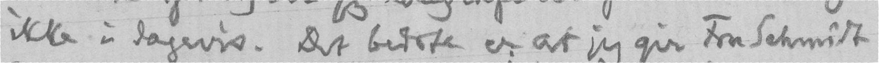ikke i dagevis. Det bedste er at jeg gir Fru Schmidt
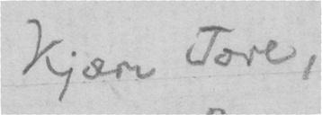Kjære Tore,
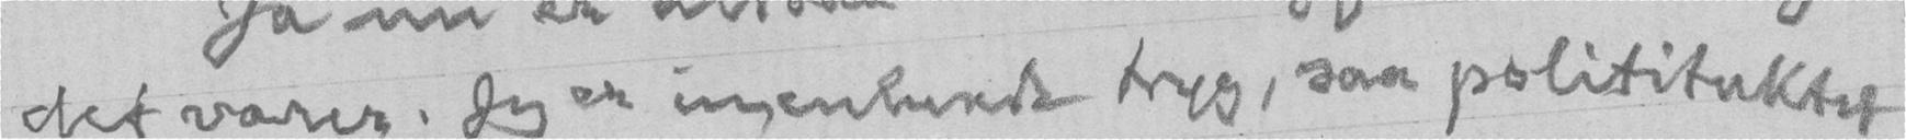det varer. Jeg er ingenlunde tryg, saa politituktet
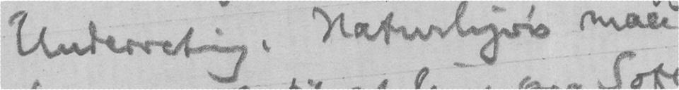Underretning. Naturligvis maae
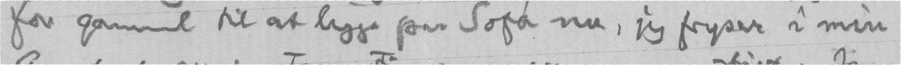for gammel til at ligge paa Sofa nu, jeg fryser i min
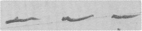- - -
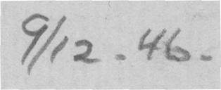9/12. 46.
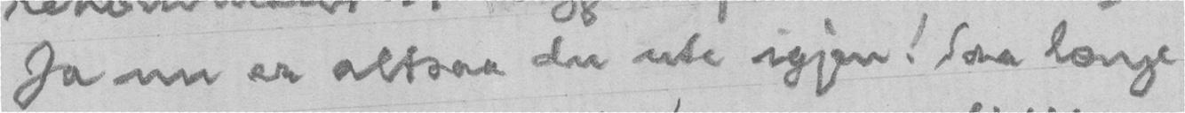Ja nu er altsaa du ute igjen! Saa længe
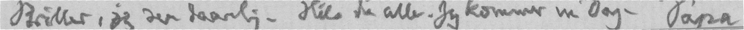Briller, jeg ser daarlig. Hils de alle. Jeg kommer en Dag. Papa
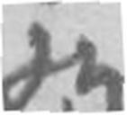jeg
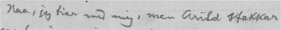Naa, jeg tier med mig, men Arild stakkar
